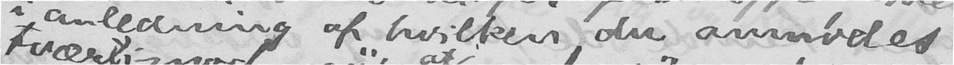i anledning af hvilken du anmodes
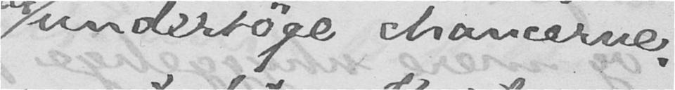undersøge chancerne.
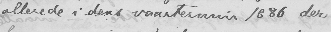allerede i dens vaartermin 1886 der
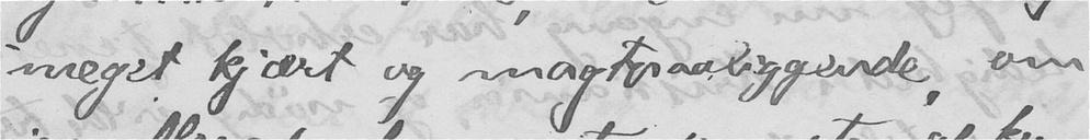meget kjært og magtpaaliggende, om
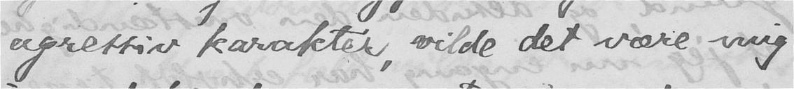agressiv karakter, vilde det være mig
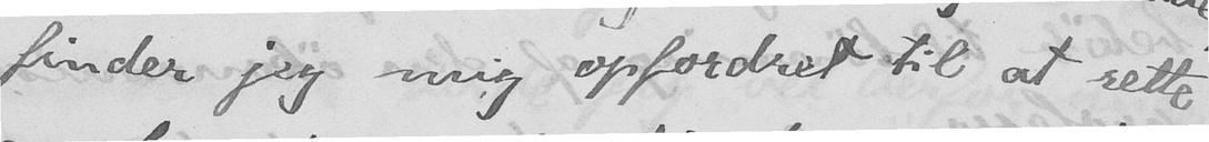finder jeg mig opfordret til at rette
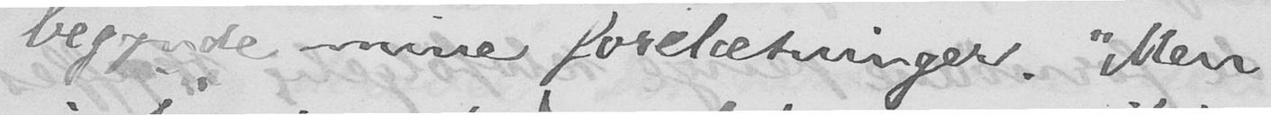begynde mine forelæsninger. "Men
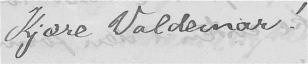Kjære Valdemar!
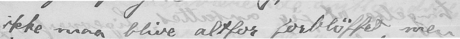ikke maa blive altfor forbløffet, men
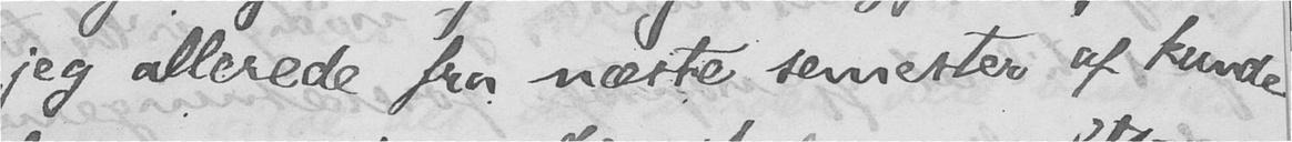jeg allerede fra næste semester af kunde
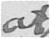at
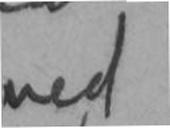ned
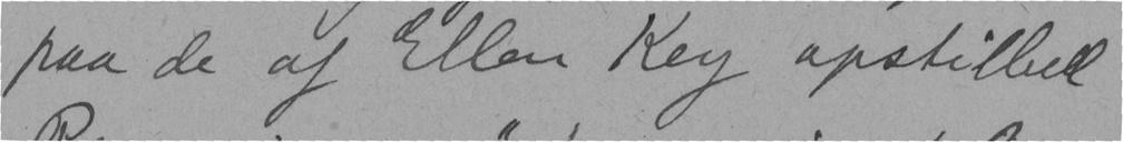paa de af Ellen Key opstillett
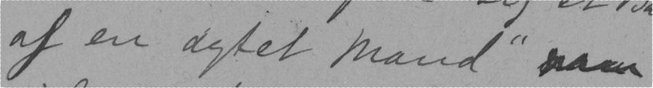af en ægtet mand"
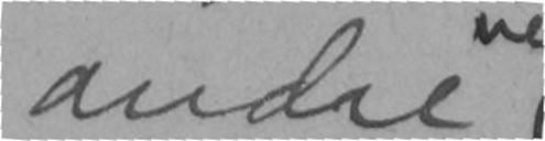andre
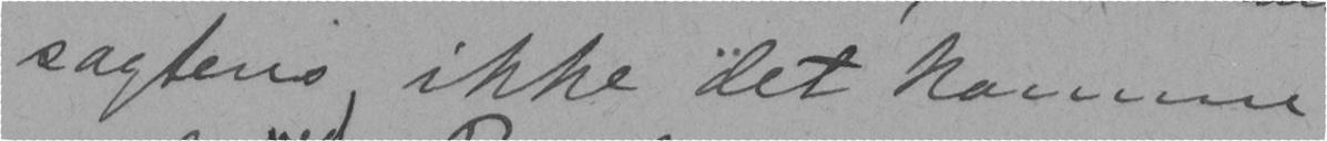sagtens ikke det komme
